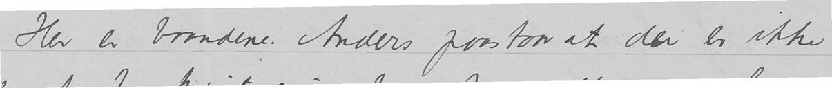Her er baandene. Anders paastaar at det er ikke
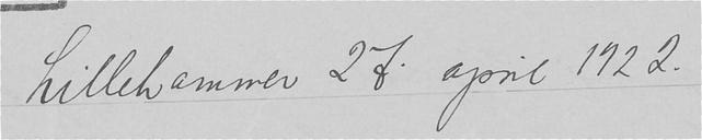Lillehammer 27. april 1922
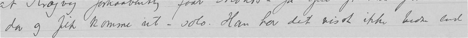da og fik komme ut – solo. Han har det visst ikke bedre end
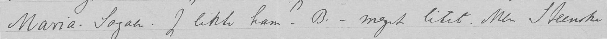Maria-Sagaen.  Jeg likte ham – B. – meget litet. Men Steenske
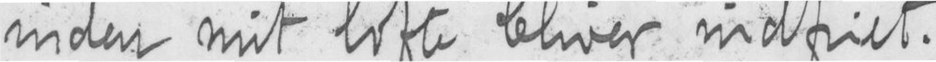inden mit løfte bliver indfriet.
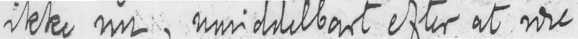ikke nu, middelbart efter at være
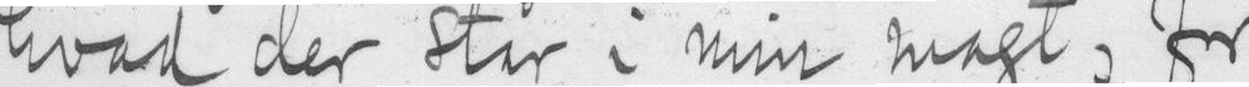hvad der står i min magt, for
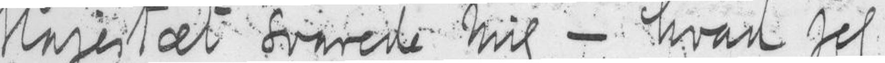Majestæt svarede mig - hvad jeg
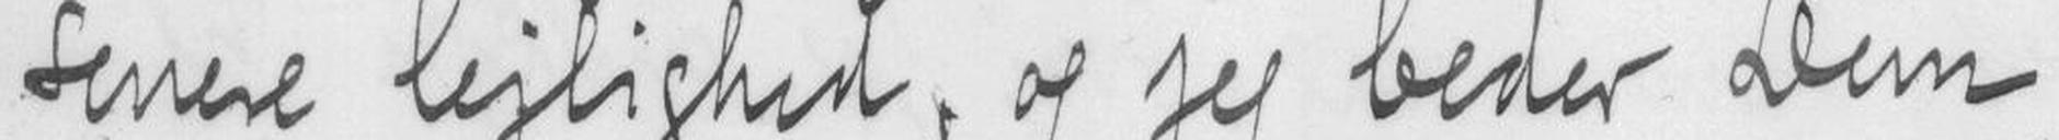senere lejlighed, og jeg beder Dem
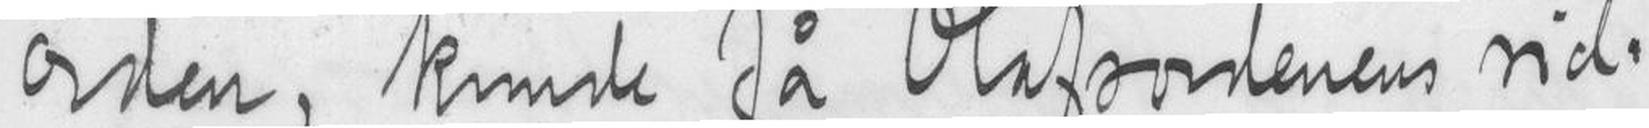orden, kunde få Olafsordenens rid-
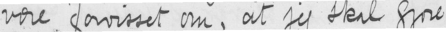være forvisset om, at jeg skal gjøre,
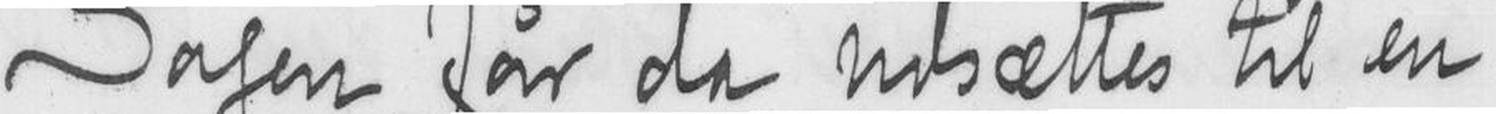Sagen får da udsættes til en
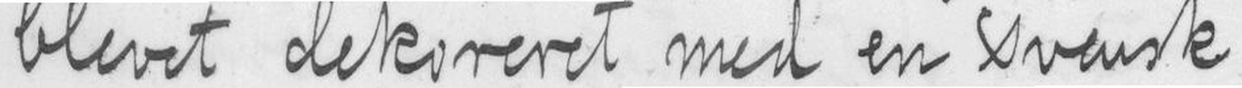blevet dekoreret med en svensk
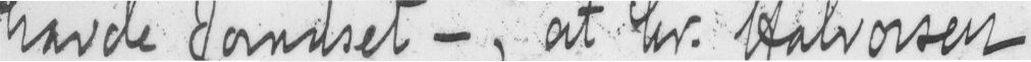havde forudset-, at hr. Halvorsen
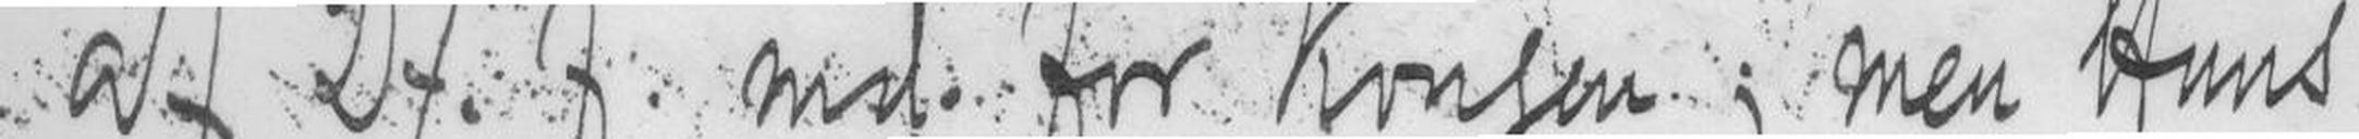af 27. f. md. for Kongen; men Hans
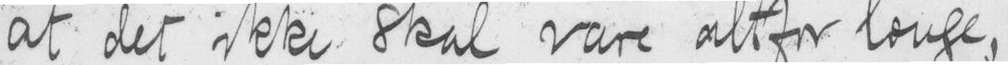at det ikke skal være altfor længe,
